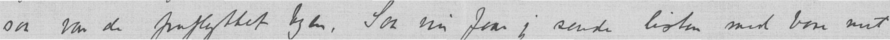saa var de fraflyttet byen, Saa nu faar jeg sende listen med bare mit
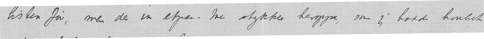listen før, men der var etpar – tre stykker heroppe, som jeg hadde haabet
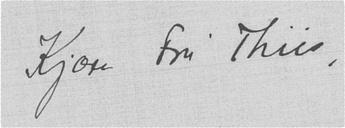Kjære Fru Thiis,
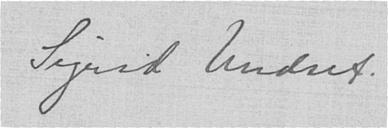Sigrid Undset.
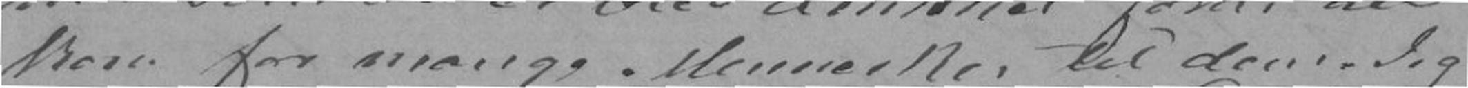kom for mange Mennesker til dem. Jeg
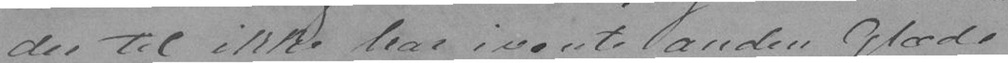der til ikke har ivente anden Glæde
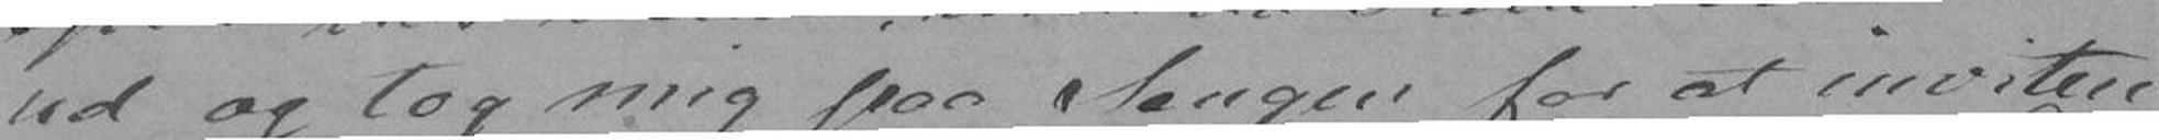ud og tog mig paa Sengen for at invitere
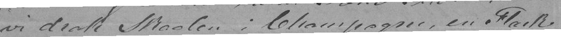vi drak Skaalen i Champagne, en Flaske
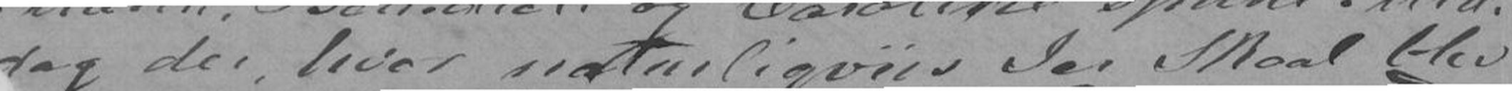dag der, hvor naturligviis Jer Skaal blev
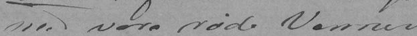med vore røde Venner,
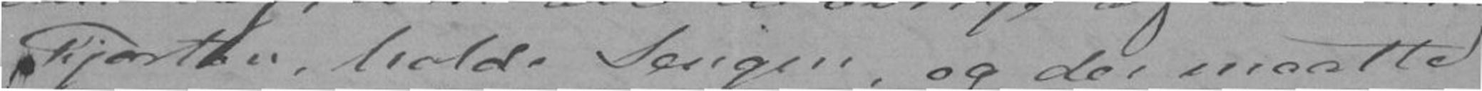Fjorten, holde Sengen, og der maatte
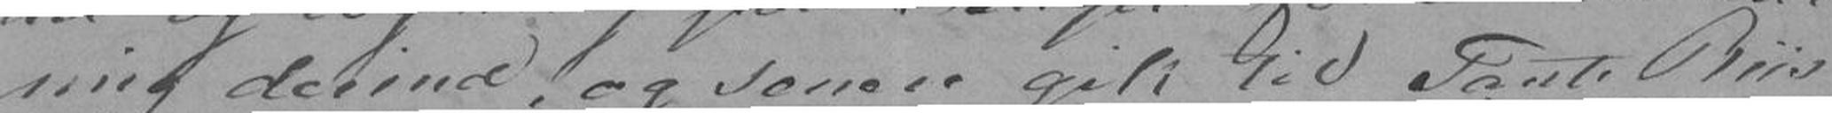mig derind, og senere gik til Tante Riis
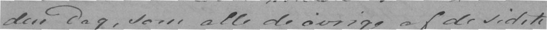den Dag, som alle de øvrige af de sidste
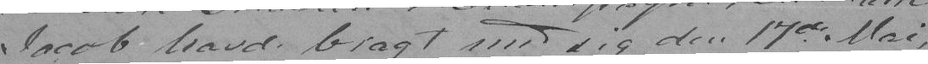Jacob havde bragt med sig den 17de Mai,
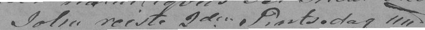John reiste 2den Pintsedag med
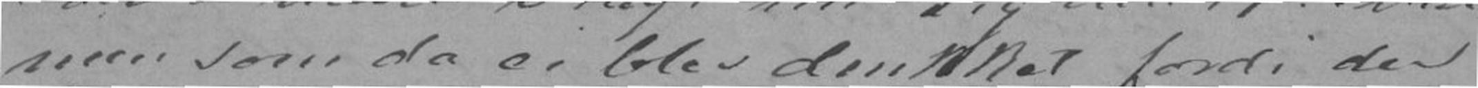men som da en blev drukket fordi der
